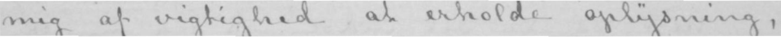mig af vigtighed at erholde oplysning,
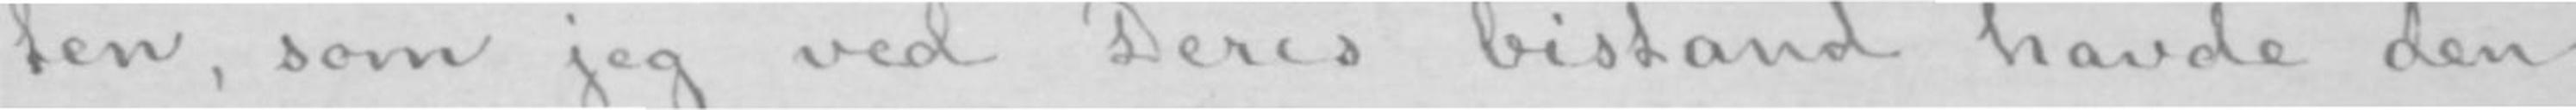ten, som jeg ved Deres bistand havde den
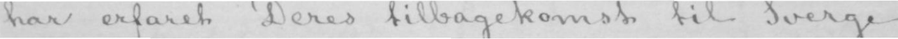har erfaret Deres tilbagekomst til Sverge,
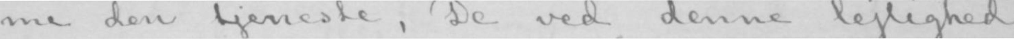me den tjeneste, De ved denne lejlighed
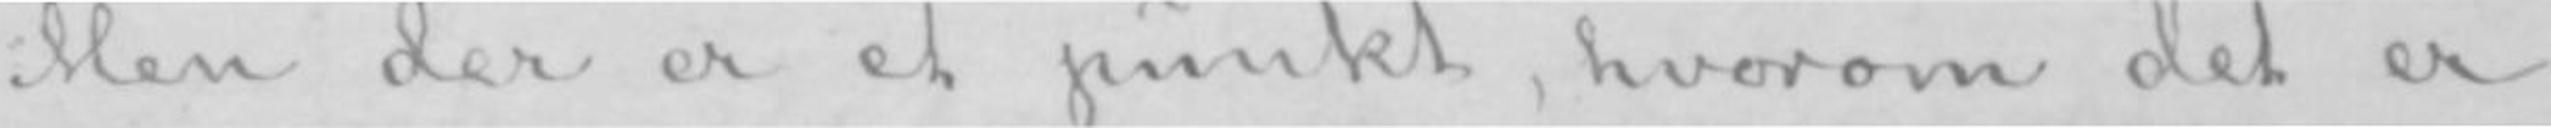Men der er et punkt, hvorom det er
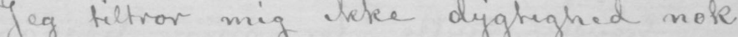Jeg tiltror mig ikke dygtighed nok
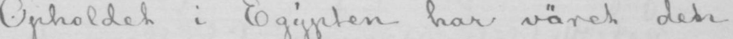Opholdet i Egypten har väret dendet
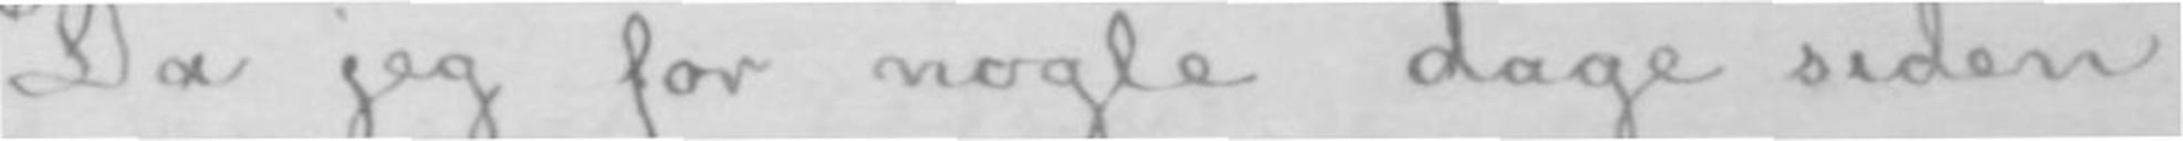Da jeg for nogle dage siden
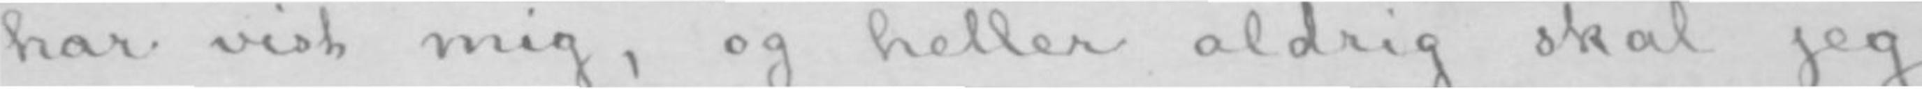har vist mig, og heller aldrig skal jeg
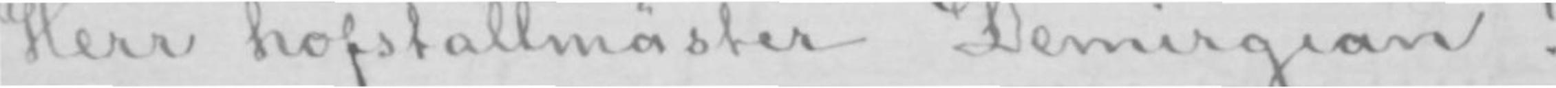Herr hofstallmäster Demirgian!
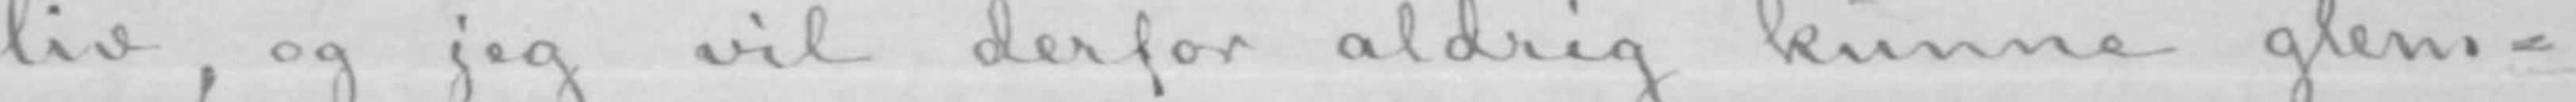liv, og jeg vil derfor aldrig kunne glem-
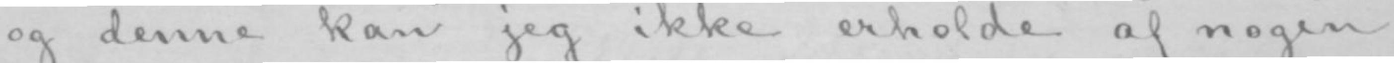og denne kan jeg ikke erholde af nogen
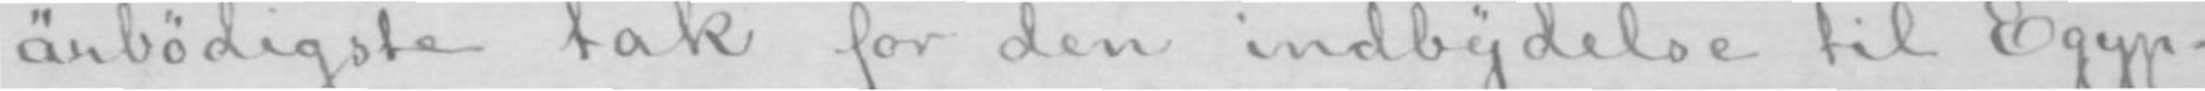ärbödigste tak for den indbydelse til Egyp-
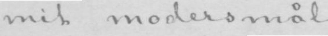mit modersmål.
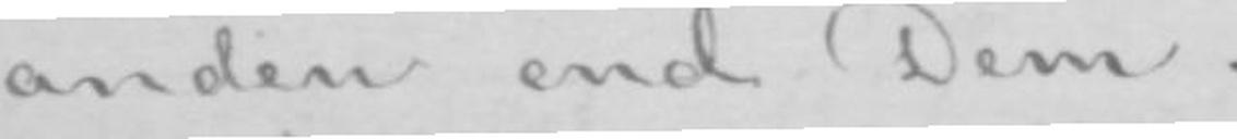anden end Dem.
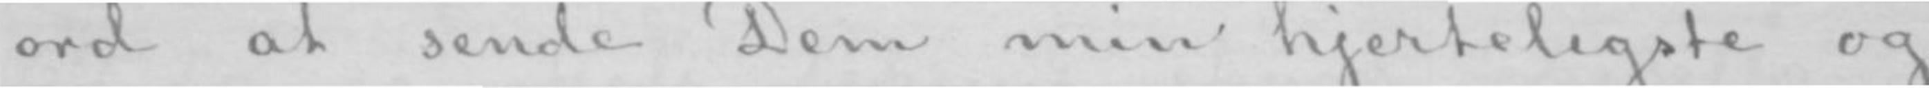ord at sende Dem min hjerteligste og
